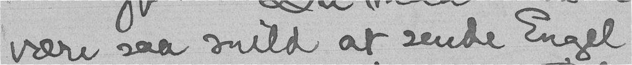være saa snild at sende Engel
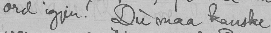ord igjen! Du maa kanske
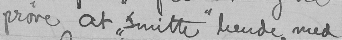prøve at "smitte" hende med
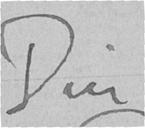Din
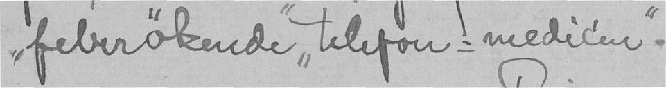"feberøkende" "telefon-medicin".
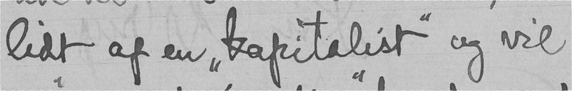lidt af en "kapitalist" og vil
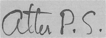Atter P. S.
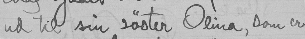ud til sin søster Olina, som er
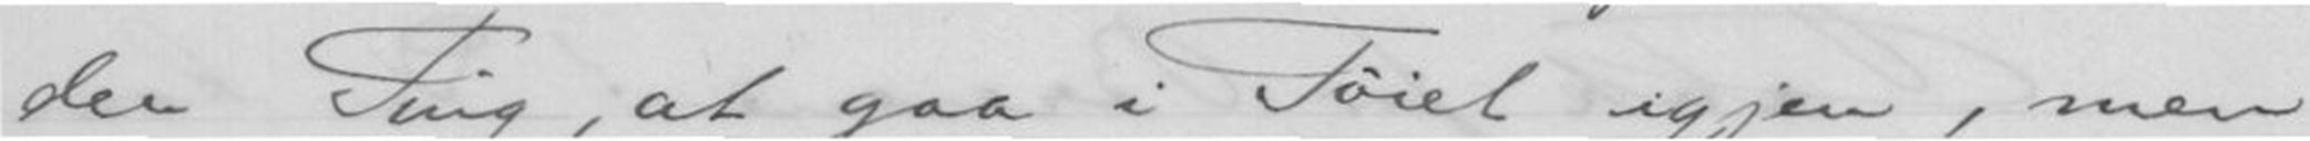den Ting, at gaa i Tøiet igjen, men
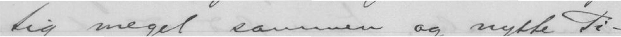tig meget sammen og nytte Ti-
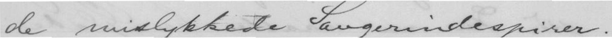de mislykkede Sangerindespirer.
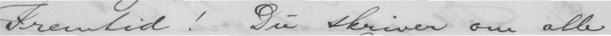Fremtid! Du skriver om alle
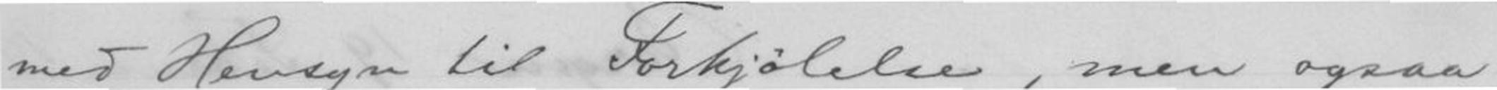med Hensyn til Forkjølelse, men ogsaa
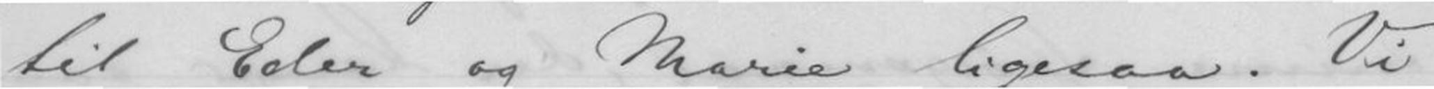til Eder og Marie ligesaa. Vi
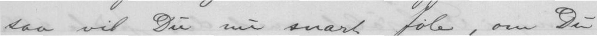saa vil Du nu snart føle, om Du
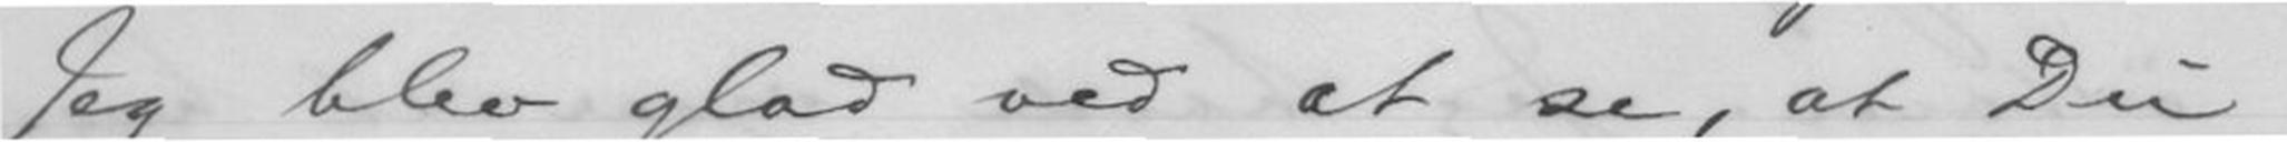Jeg blev glad ved at se, at Du
